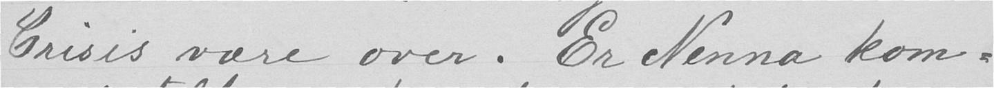Crisis være over. Er Nenna kom-
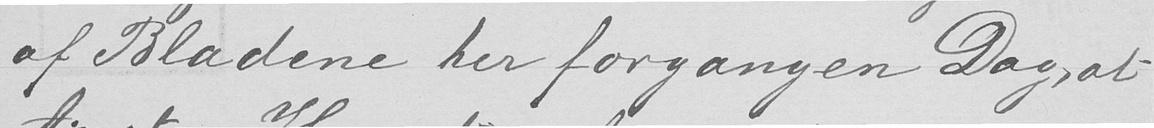af Bladene her forgangen Dag, at
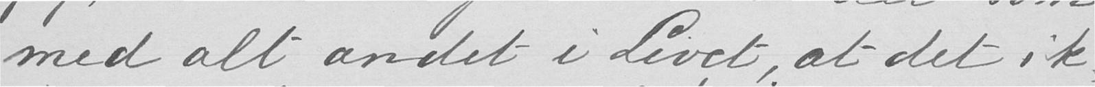med alt andet i Livet, at det ik-
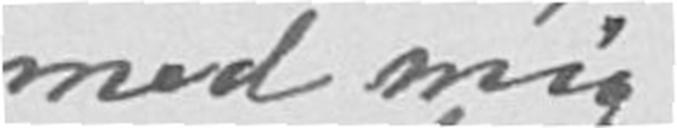med mig
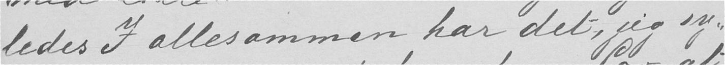ledes I allesammen har det, jeg sy-
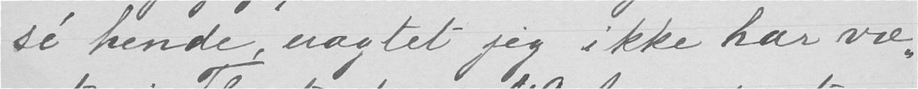sé hende, uagtet jeg ikke har væ-
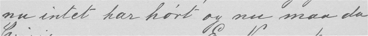nu intet har hørt og nu maa da
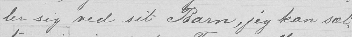ler sig ved sit Barn, jeg kan sæt-
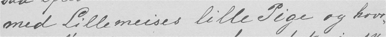med Lillemeises lille Pige og hvor-
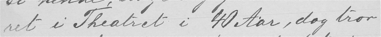ret i Theatret i 40 Aar, dog tror
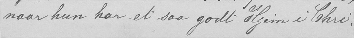naar hun har et saa godt Hjem i Chri-
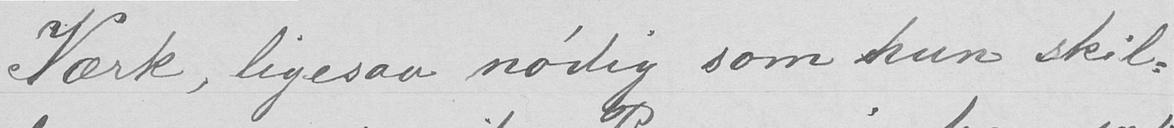Værk, ligesaa nødig som kun skil-
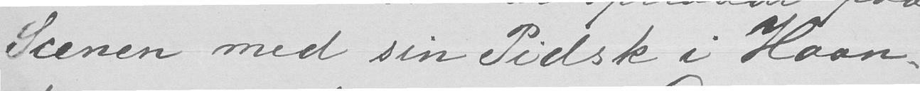Scenen med sin Pidsk i Haan-
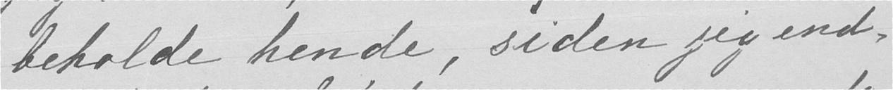beholde hende, siden jeg end-
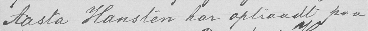Aasta Hansten har optraadt paa
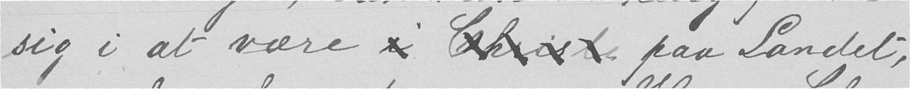sig i at være  paa Landet,
